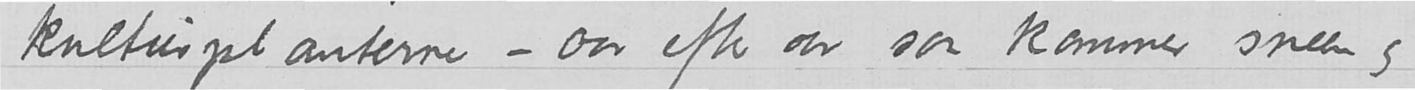kulturplanterne – aar efter aar saa kommer sneen og
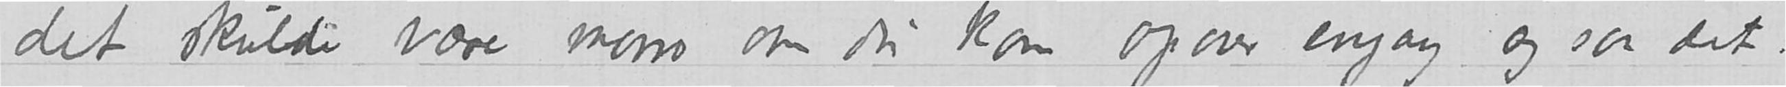det skulde være morro om du kom opover engang og saa det.
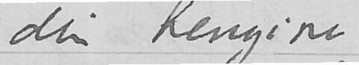din hengivne
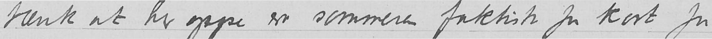tænk at her oppe er sommeren faktisk for kort for
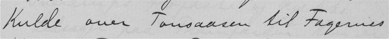Kulde over Tonsaasen til Fagernes
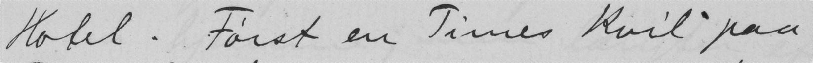Hotel. Først en Times Kvil paa
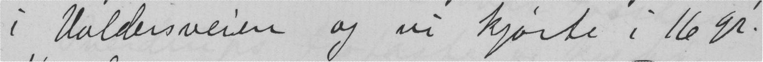i Valdersveien og vi kjørte i 16 gr.
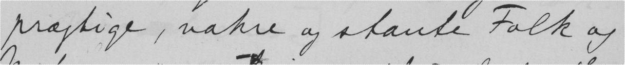prægtige, vakre og staute Folk og
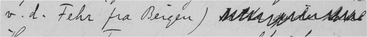v.d. Fehr fra Bergen)
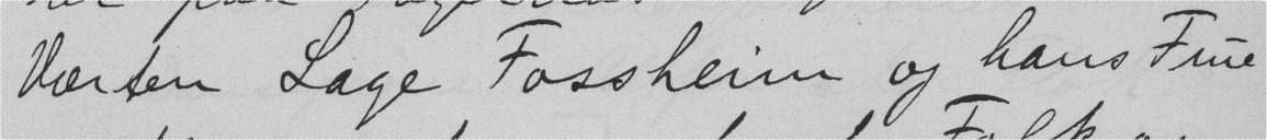Værten Lage Fossheim og hans Frue
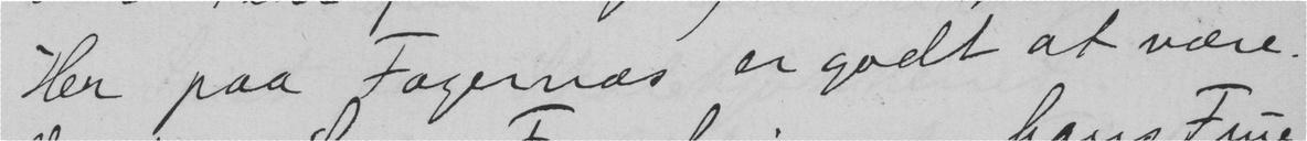Her paa Fagernæs er godt at være.
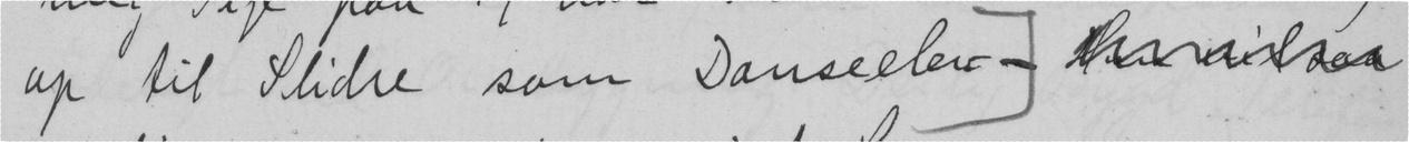op til Slidre som Danseelev -
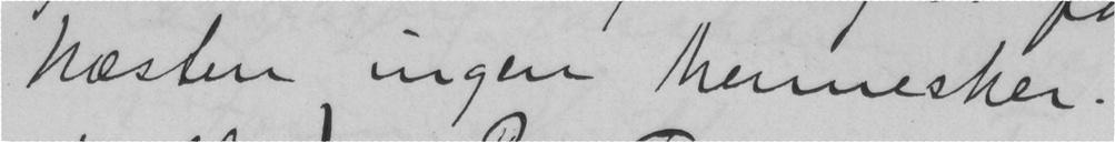Næsten ingen Mennesker.
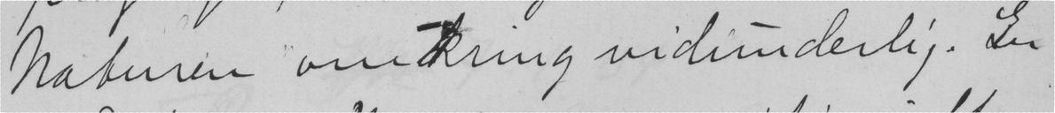Naturen omkring vidunderlig. En
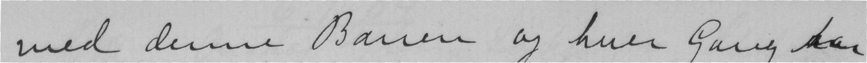med denne Banen og hver Gang har
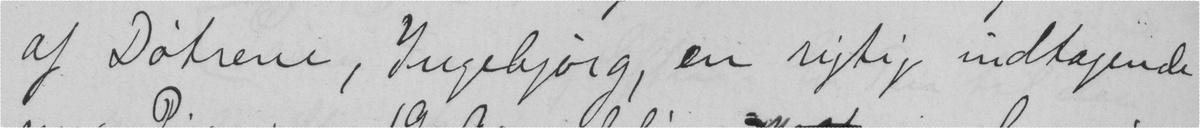af Døtrene, Ingebjørg, en rigtig indtagende
